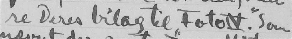se Deres bilag til "FotoIV". Som
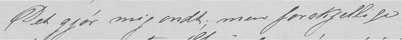Det gjør mig ondt; men forskjellige
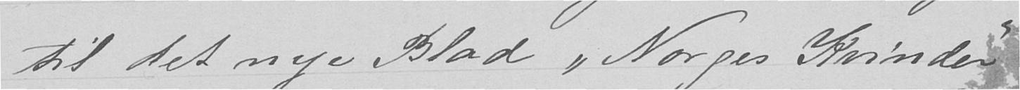til det nye Blad "Norges Kvinder"
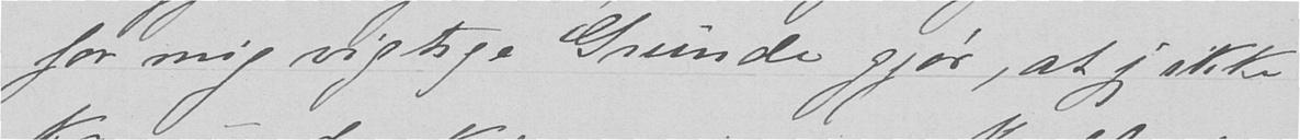for mig vigtige Grunde gjør, at jeg ikke
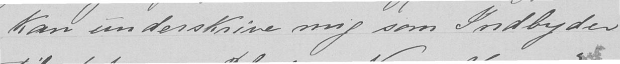kan underskrive mig som Indbyder
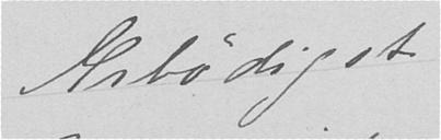Ærbødigst
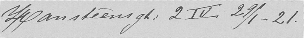Hansteensgt. 2 IV  24/1 - 21.
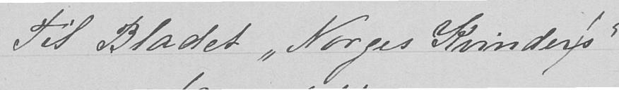Til Bladet "Norges Kvinders"
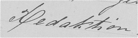Redaktion
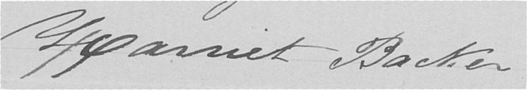Harriet Backer.
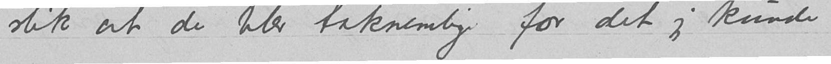slik at de blev taknemlige for det jeg kunde
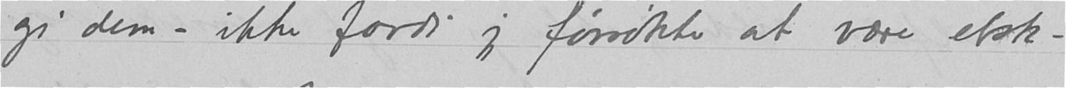gi dem – ikke fordi jeg forsøkte at være elsk-
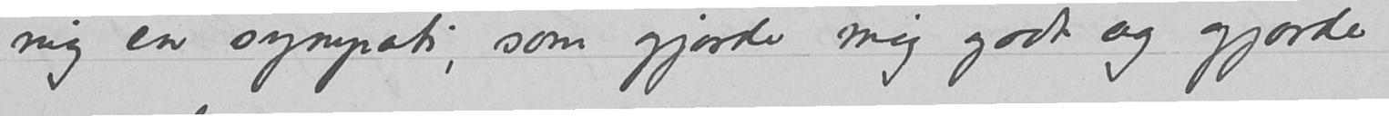mig en sympati, som gjorde mig godt og gjorde
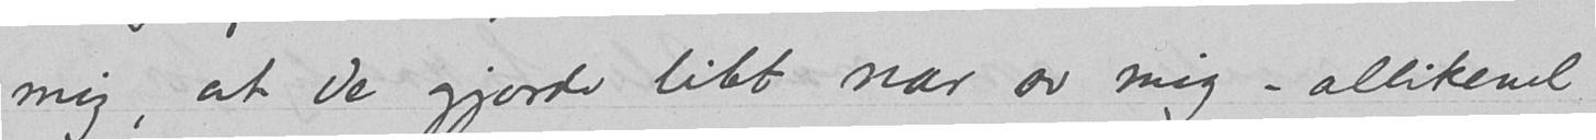mig, at De gjorde litt nar av mig - allikevel
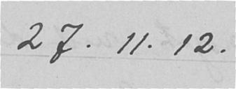27.11.12.
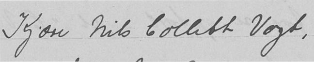Kjære Nils Collett Vogt,
